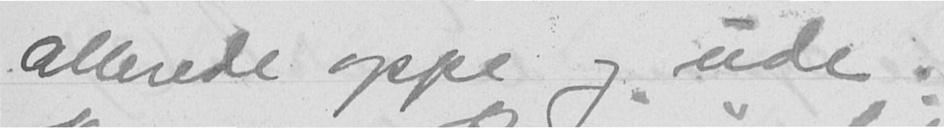allerede oppe og ude.
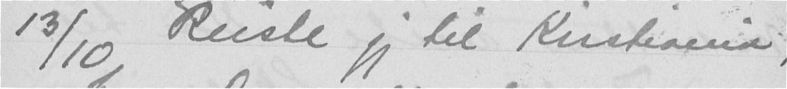13/10 Reiste jeg til Kristiania,
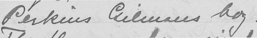Perkins Gilmans bog:
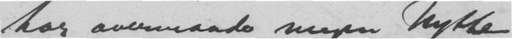har overmaade megen Nytte
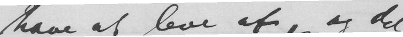have at leve af, og det
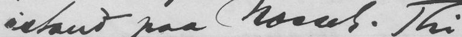istand paa Næsset. Thi
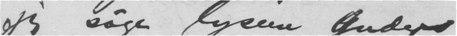jeg søge lysere Guder
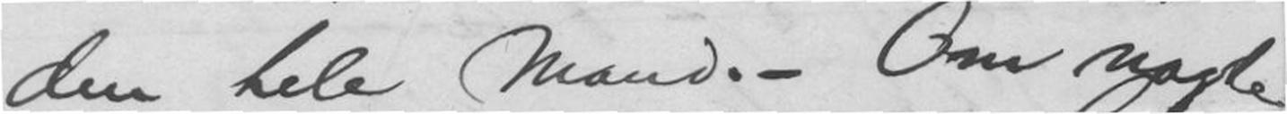den hele Mand. - Om nogle
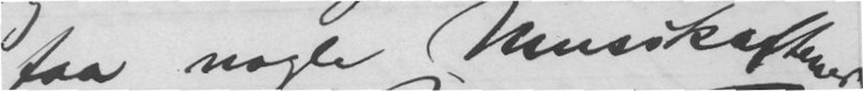faa nogle Musikaftener
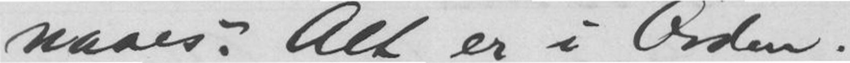naaes. Alt er i Orden.
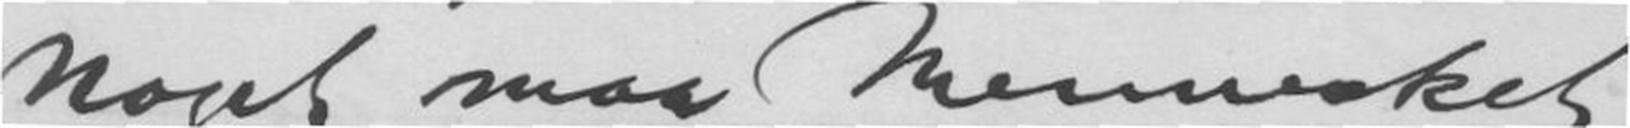noget maa Mennesket
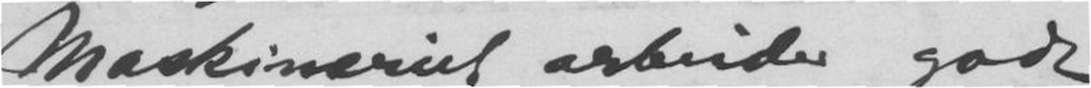Maskineriet arbeider godt
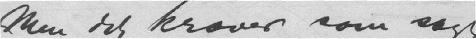Men det kræver som sagt
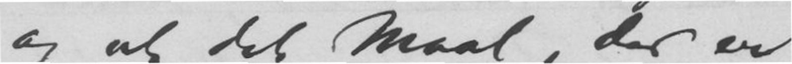og at det Maal, der er
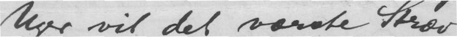Uger vil det værste Stræv
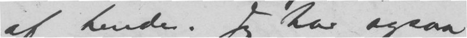af hende. Jeg har ogsaa
